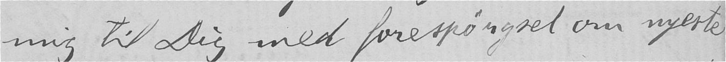mig til Dig med forespørgsel om nyeste
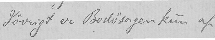Iøvrigt er Bodøsagen kun af
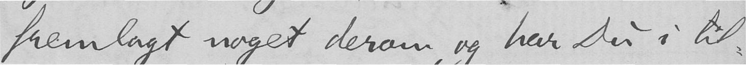fremlagt noget derom, og har Du i til-
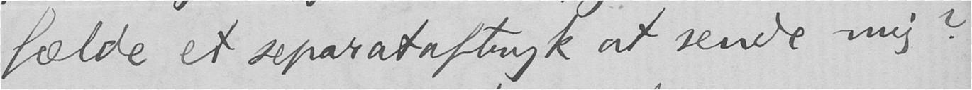fælde et separataftryk at sende mig?
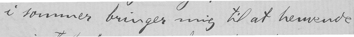i sommer bringer mig til at henvende
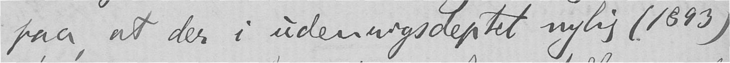paa, at der i udenrigsdeptet nylig (1893)
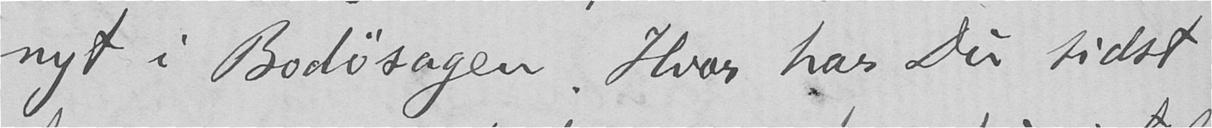nyt i Bodøsagen. Hvor har Du sidst
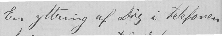En yttring af Dig i telefonen
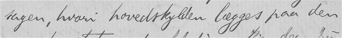sagen, hvori hovedskylden lægges paa den
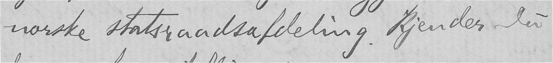norske statsraadsafdeling. Kjender Du
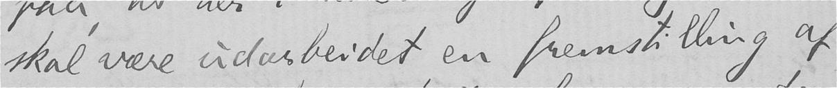skal være udarbeidet en fremstilling af
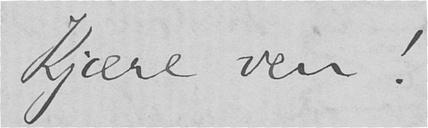Kjære ven!
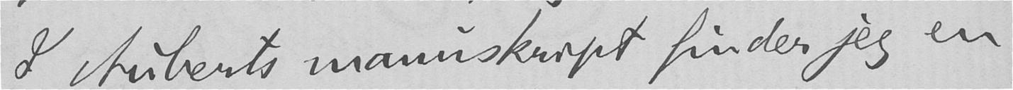I Auberts manuskript finder jeg en
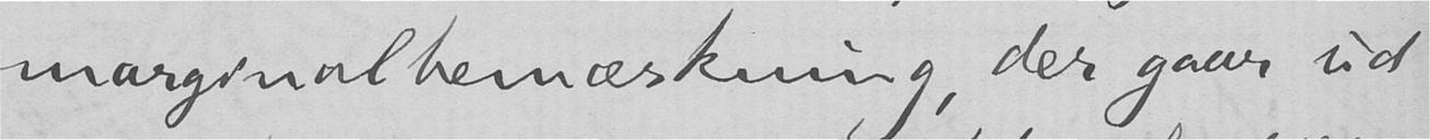marginal bemærkning, der gaar ud
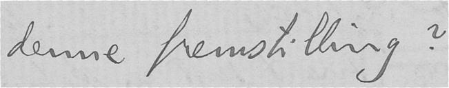denne fremstilling?
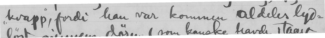"kvapp", fordi han var kommen aldeles lyd-
